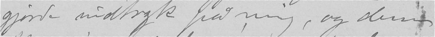gjorde indtryk på mig, og dem
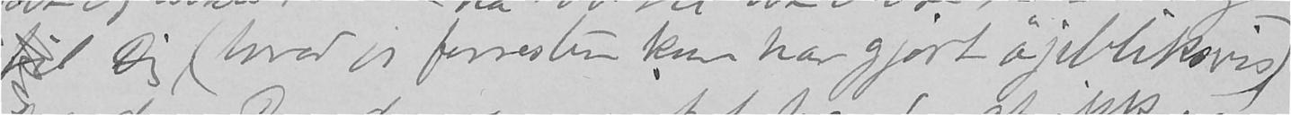til Dig (hvad jeg forresten kun har gjort øjebliksvis)
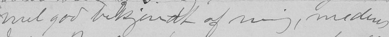mel god bekjendt af mig, medens
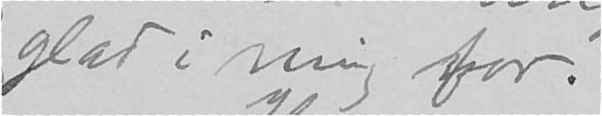glad i mig for.
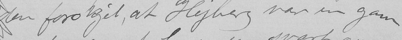den forskjel, at Hejberg var en gam-
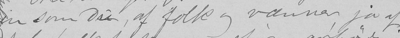en som Du, af folk og vænner, ja af
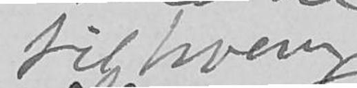til hvem
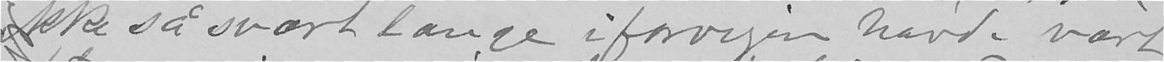ikke så svært længe iforvejen havde vært
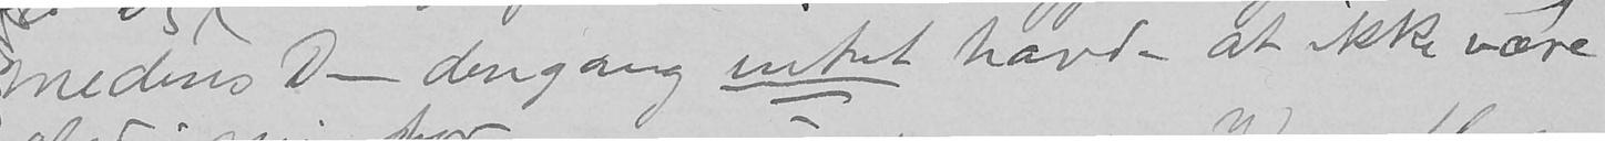medens De dengang intet havde at ikke være
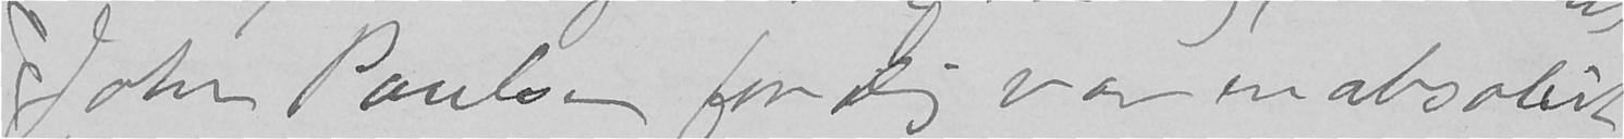John Paulsen for dig var en absolut
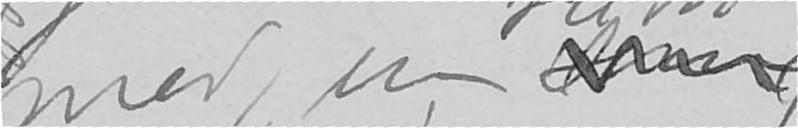med, en som
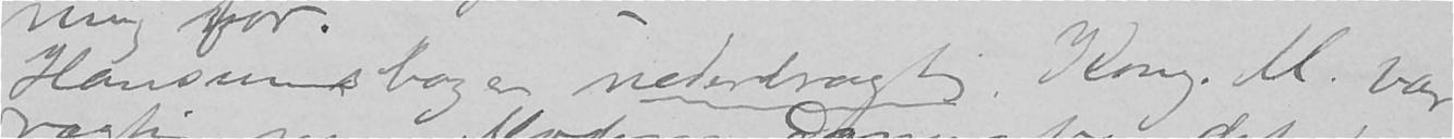Hamsuns bog er nederdrægtig. Kong M. var
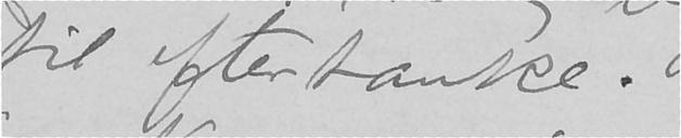til eftertanke.
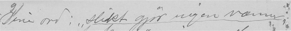Dine ord: "Slikt gjør ingen vænner",
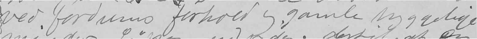ved fordums forhold og gamle hyggelige
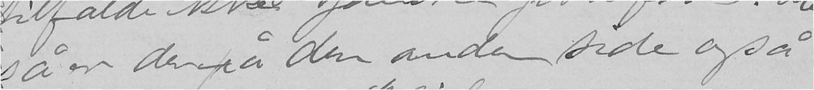så er der på den anden side også
